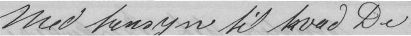Med hensyn til hvad De
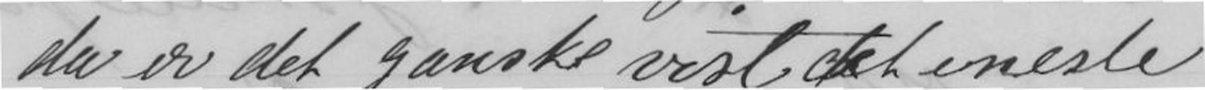da er det ganske vist det eneste
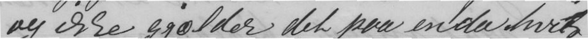og ikke gjælder det paa enda hvis
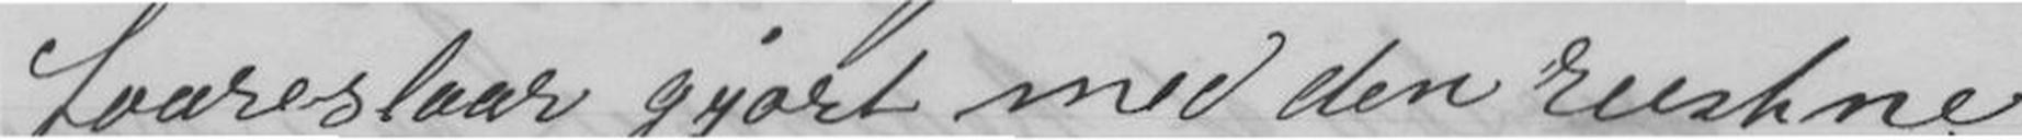faareslaar gjort med den rustne
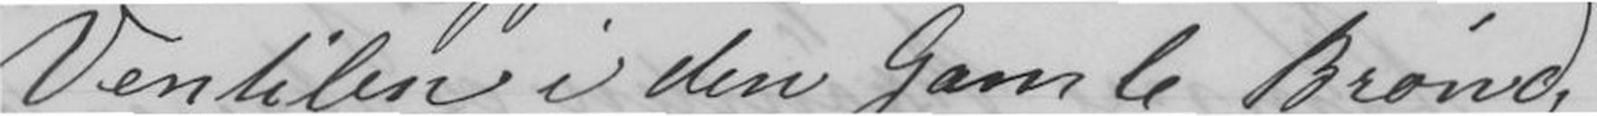Ventilen i den Gamle Brønd,
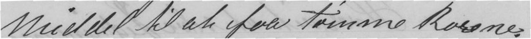Middel til at faa tømme Rørene.
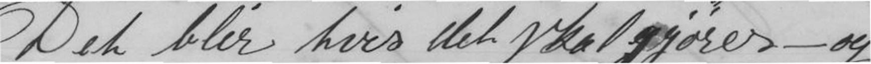Det blir hvis det skal gjøres - og
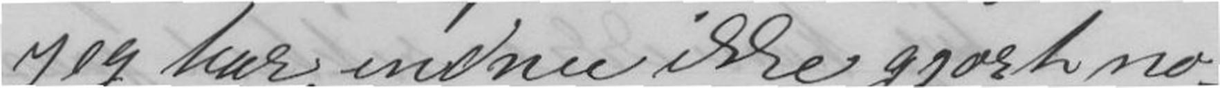Jeg har endnu ikke gjort no-
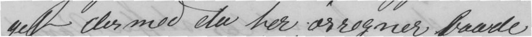get dermed da her øsregnet baade
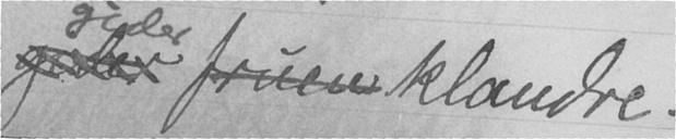gider fruen klandre.
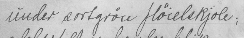under sortgrøn fløielskjole;
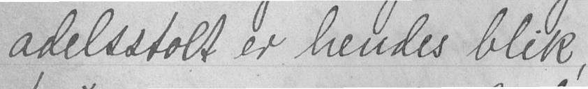adelsstolt er hendes blik,
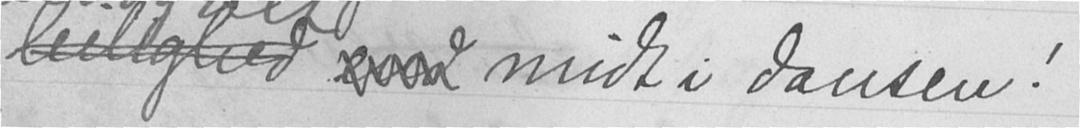leilighed end midt i dansen!
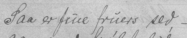Saa er fine fruers sed, -
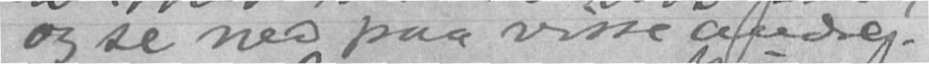og se ned paa visse andre.
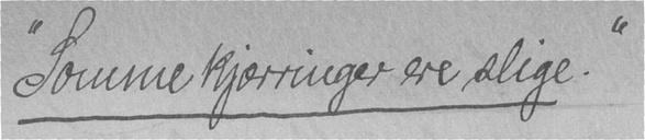"Somme kjærringer ere slige."
